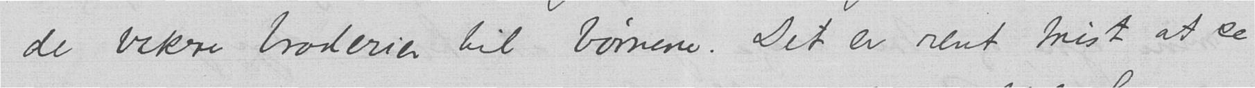de vakre broderier til børnene. Det er rent trist at se
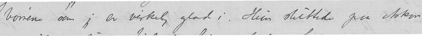børnene som jeg er virkelig glad i. Hun sluttede paa Askov
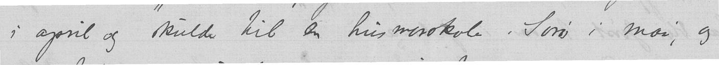i april og skulde til en husmorskole i Sorø i mai, og
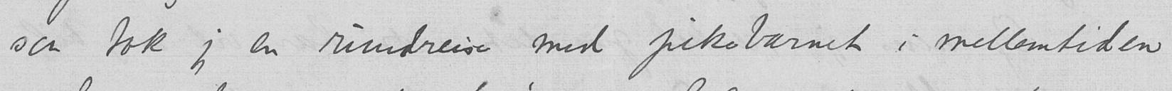saa tok jeg en rundreise med pikebarnet i mellemtiden
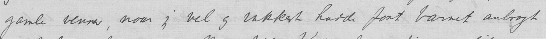gamle venner, naar jeg vel og vakkert hadde faat barnet anbragt
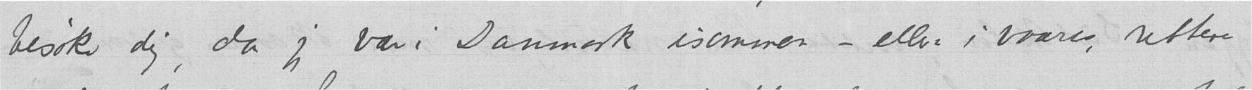besøke dig, da jeg var i Danmark isommer - eller ivaares, rettere
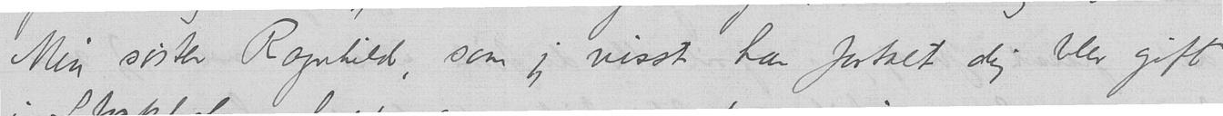Min søster Ragnhild, som jeg visst har fortalt dig blev gift
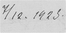7/12 - 1923.
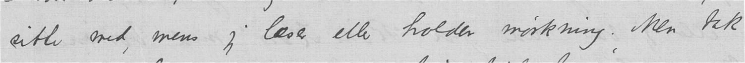sitte med, mens jeg leser eller holder mørkning. Men tak
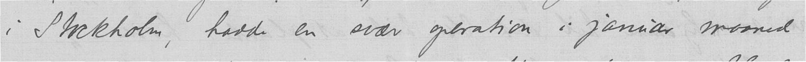i Stockholm, hadde en svær operation i januar maaned
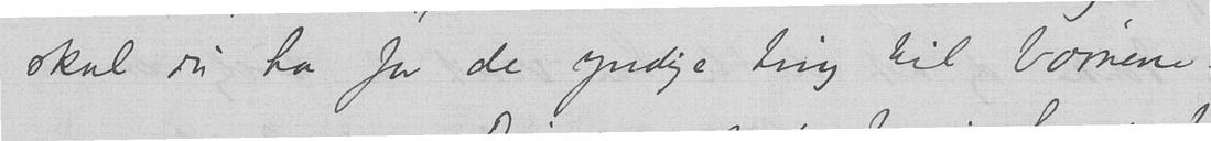skal du ha for de yndige ting til børnene.
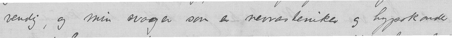vendig, og min svoger som er nevrosteniker og hypokonder
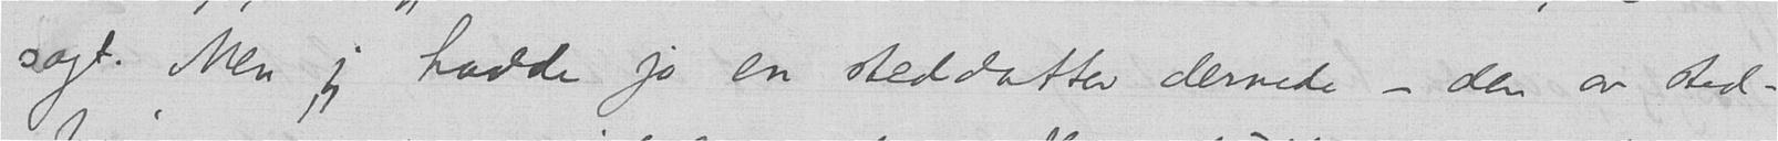sagt. Men jeg hadde jo en steddatter dernede - den av sted-
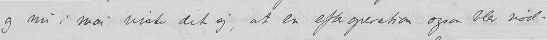og nu i mai viste det sig, at en efteroperation ogsaa blev nød-
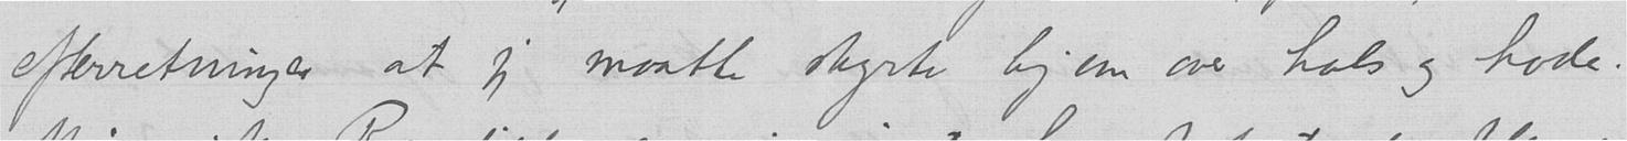efterretninger at jeg maatte styrte hjem over hals og hode.
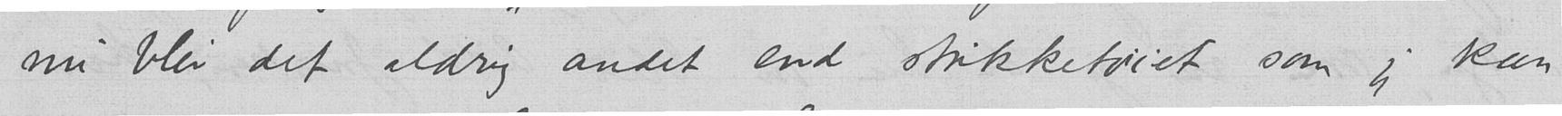- nu blir det aldrig andet end strikketøiet som jeg kan
